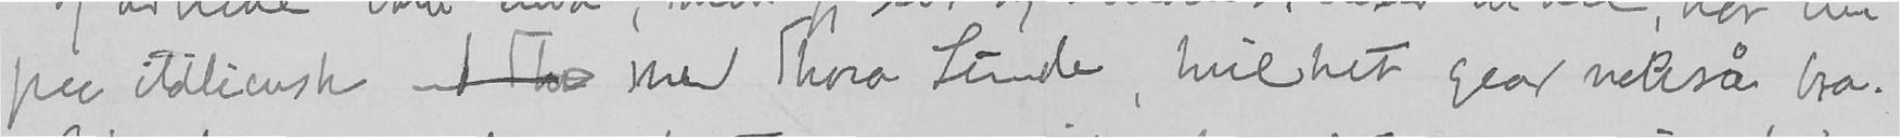paa italiensk d Tho med Thora Linde, hvilket gaar nokså bra.
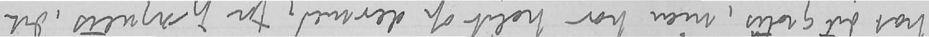hat det gratis, men har holdt op dermed, for jeg syntes, det
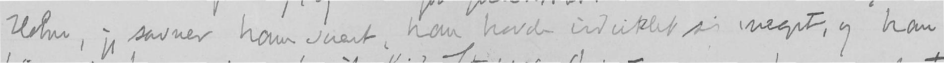Holm, jeg savner ham svært, han havde udviklet sig meget, og han
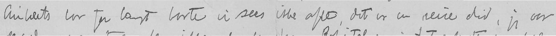Auberts bor for langt borte vi sees ikke ofte, det er en reise did, jeg var
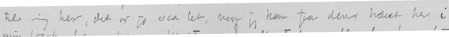til mig her, det er jo saa let, naar jeg kan faa den hævet her i
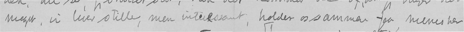meget, vi lever stille, men interessant, holder os sammen faa mennesker
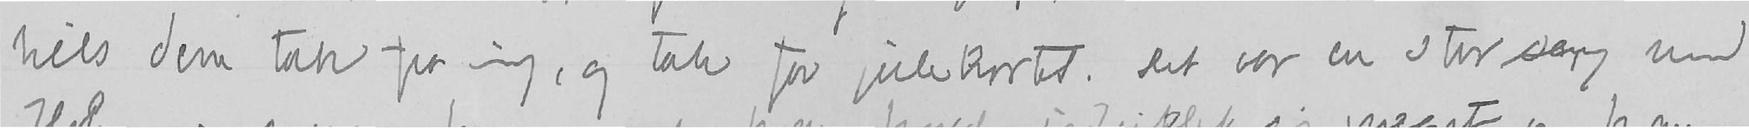hils dem tak fra mig, og tak for julekortet. Det var en stor sorg med
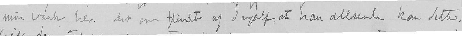min bank her. Det var flinkt af Ingolf, at han allerede kan dette,
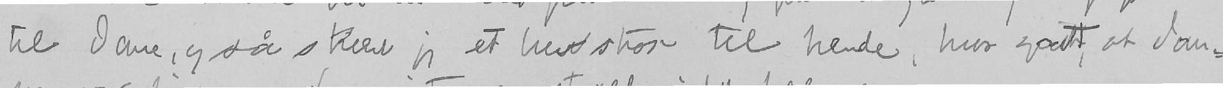til Jane, og så skrev jeg et brev strax til hende, hvor godt, at Jan-
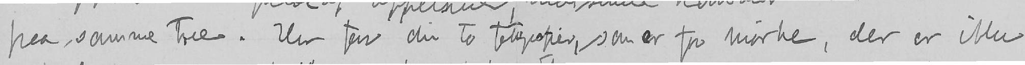paa samme træ. Her faar du to fotografier, som er for mørke, der er ikke
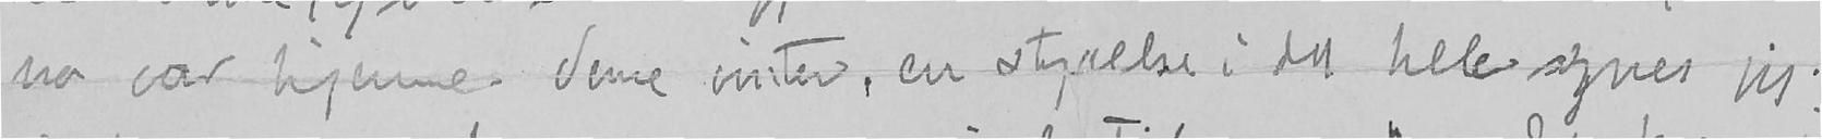na var hjemme denne vinter, en styrelse i det hele synes jeg.
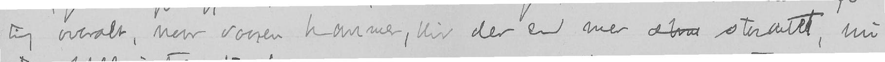ting overalt, naar vaaren kommer, blir der end mer storartet, nu
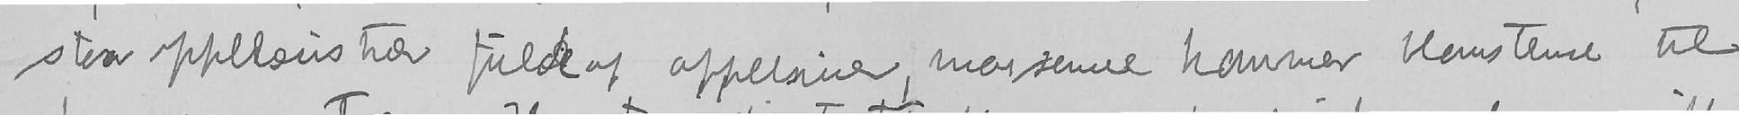staar appelsintrær fulde af appelsiner, men senere kommer blomsterne til
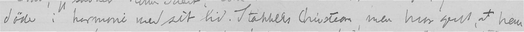døde i harmoni med sit liv. Stakkels Christian, men hvor godt, at han
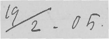19/2 - 05.
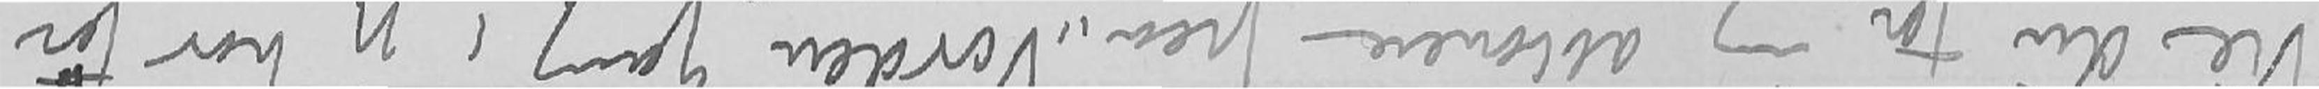Vil du for mig abbonere paa «Verdens Gang», jeg har før
